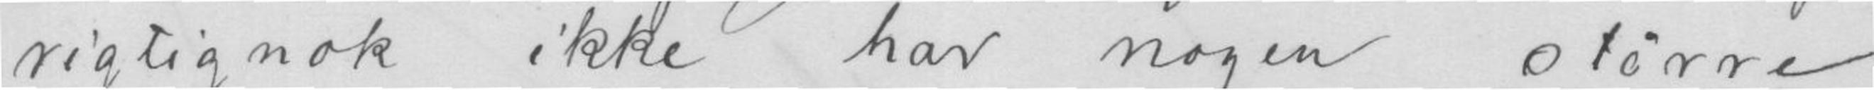rigtignok ikke har nogen større
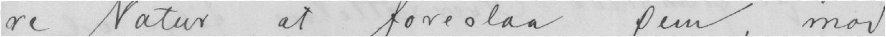re Natur at foreslaa Dem, med
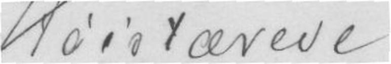Høistærede
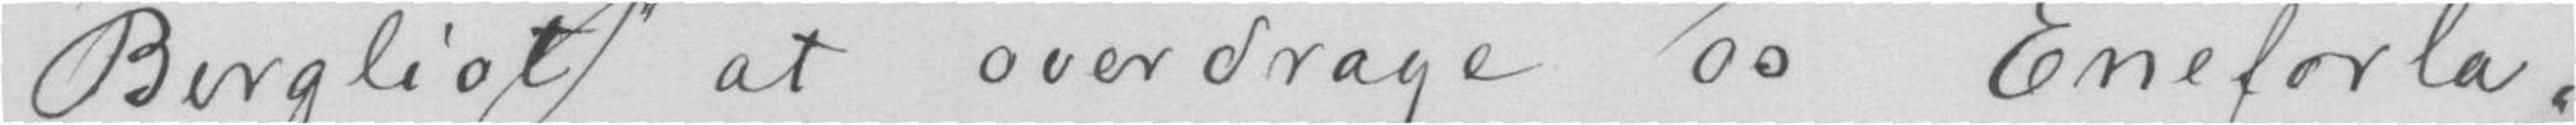Bergliot at overdrage os Eneforla-
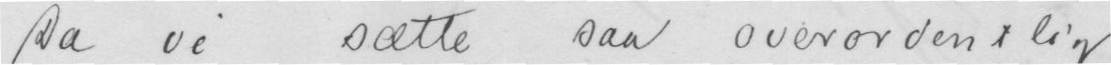Da vi sætte saa overordentlig
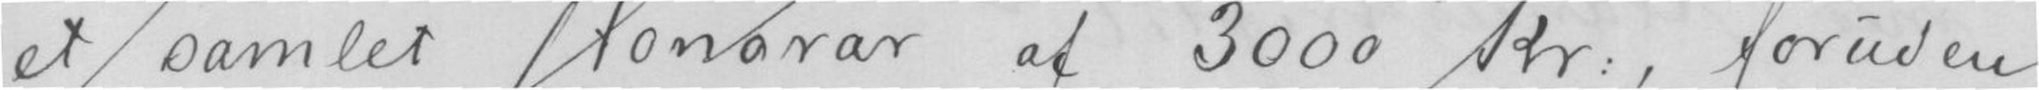et samlet Honorar Af 3000 Kr:, foruden
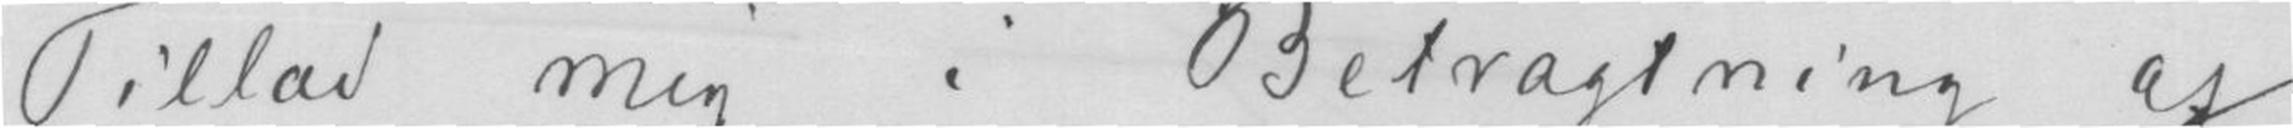Tillad mig i Betragtning af
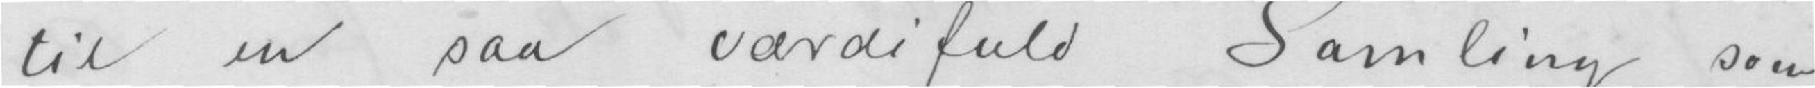til en saa værdigfuld Samlig som
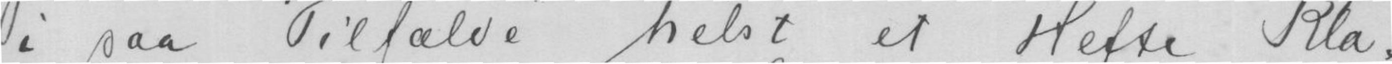i saa Tilfælde helst et Hefte Kla-
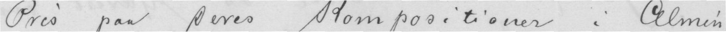Pris paa Deres Kompositioner i Almin-
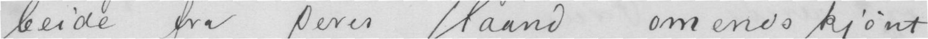beide fra Deres haand, omendskjønt
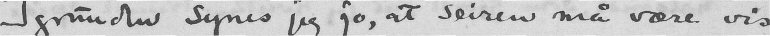I grunden synes jeg jo, at seiren må være vis
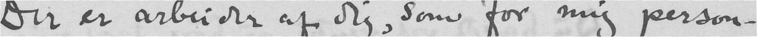Der er arbeider af dig, som for mig person-
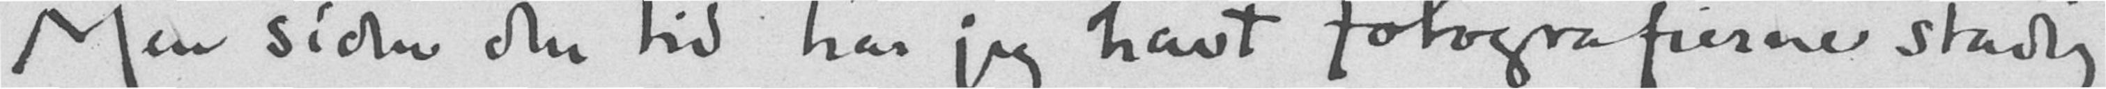Men siden den tid har jeg havt fotografierne stadig
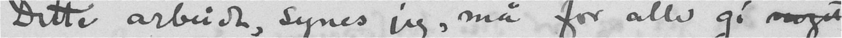Dette arbeide, synes jeg, må for alle gi noget
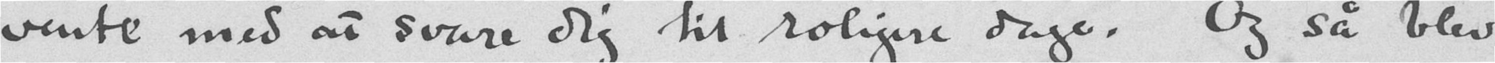vente med at svare dig til roligere dage. Og så blev
